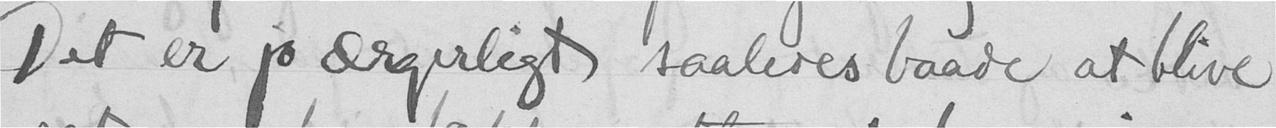Det er jo ærgerligt saaledes baade at blive
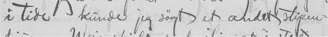i tide kunde jeg søgt et andet stipen-
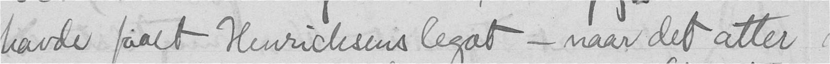havde faaet Henrichsens legat - naar det atter
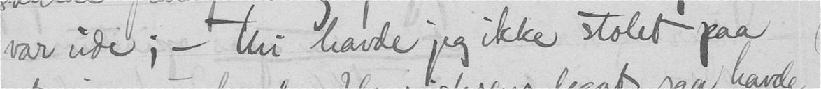var ude; - thi havde jeg ikke stolet paa
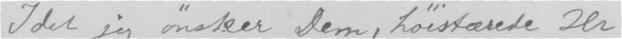Idet jeg ønsker Dem, høistærede Hr
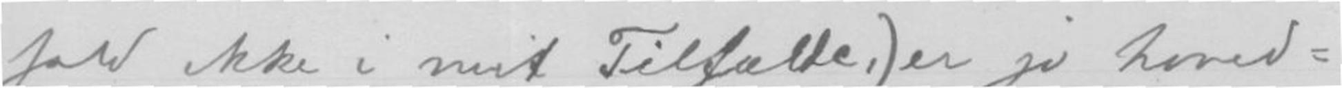fald ikke i mit Tilfælde,) er jo hoved-
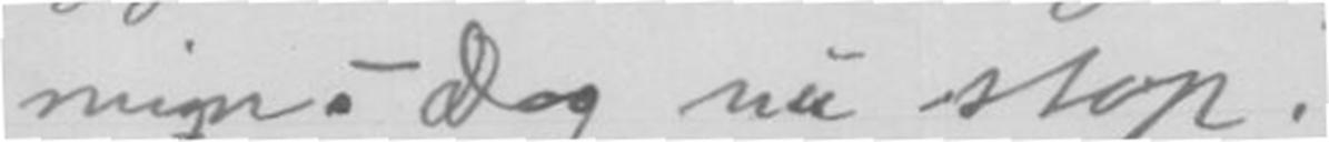min. - Dog nu stop! -
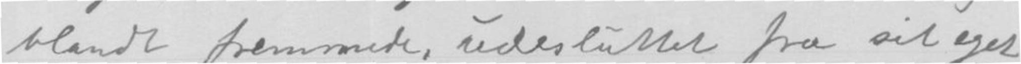blandt fremmede, udesluttet fra sit eget
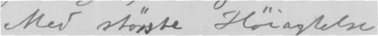Med største Høiagtelse
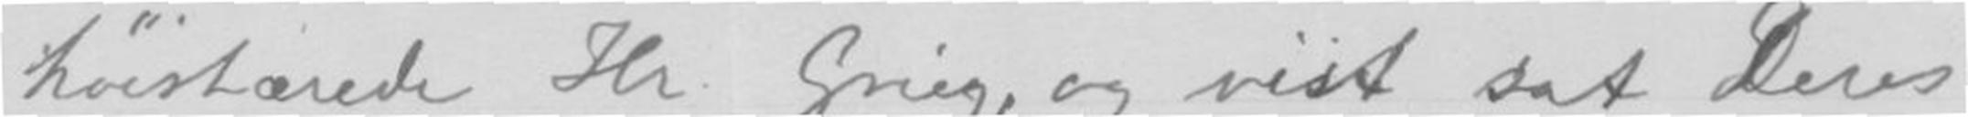høistærede Hr Grieg, og vist sat Deres
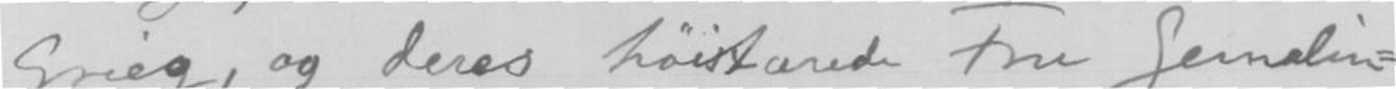Grieg, og deres høitærede Fru Gemalin-
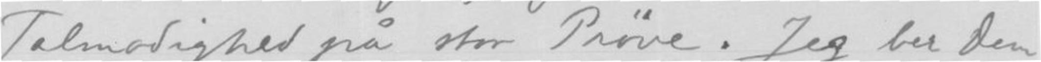Tolmodighed på stor Prøve. Jeg ber Dem
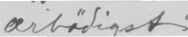ærbøidigst
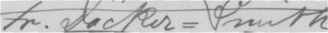Fr. Döcker=Smith.
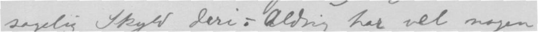sagelig Skyld Deri. - Aldrig har vel nogen
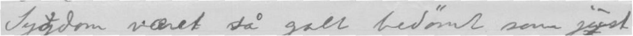Sygdom været så galt bedømt som just
